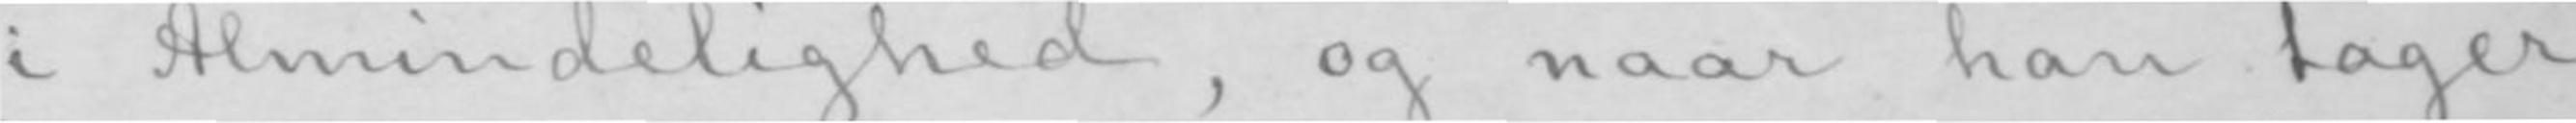i Almindelighed, og naar han tager
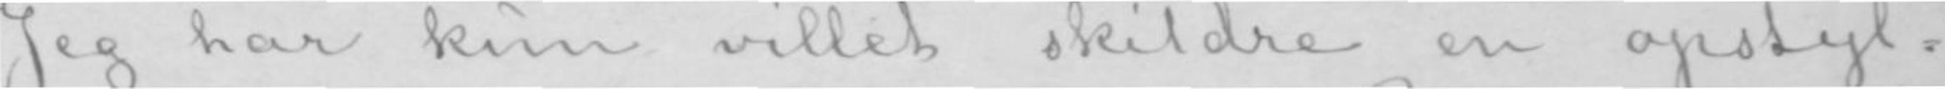Jeg har kun villet skildre en opstyl-
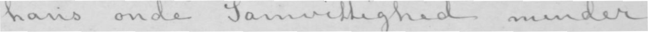hans onde Samvittighed minder
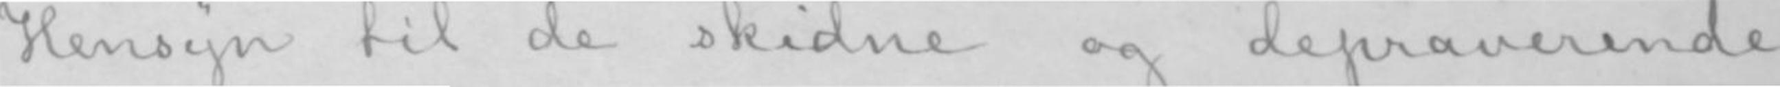Hensyn til de skidne og depraverende
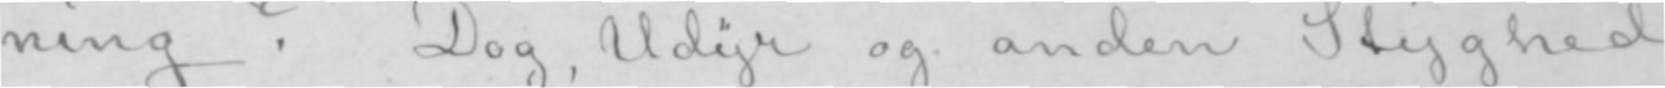ning? Dog, Udyr og anden Styghed
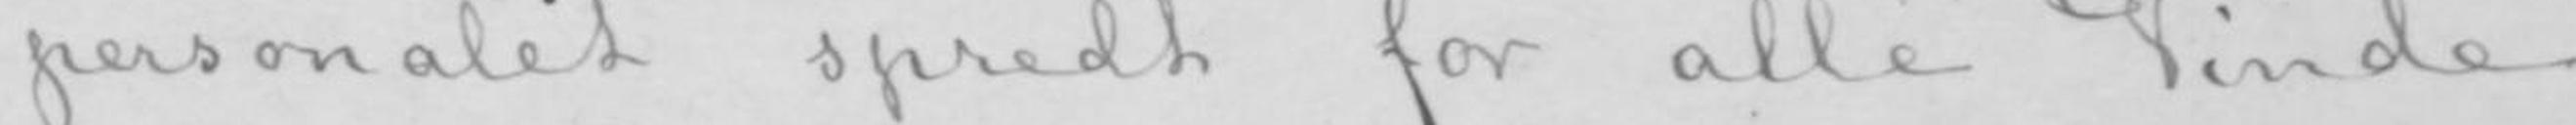personalet spredt for alle Vinde.
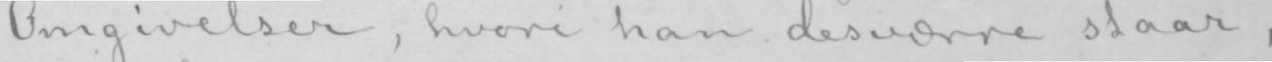Omgivelser, hvori han desværre staar,
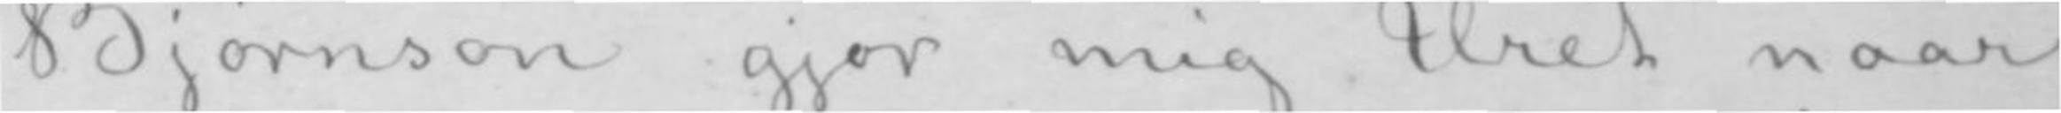Bjørnson gjør mig Uret naar
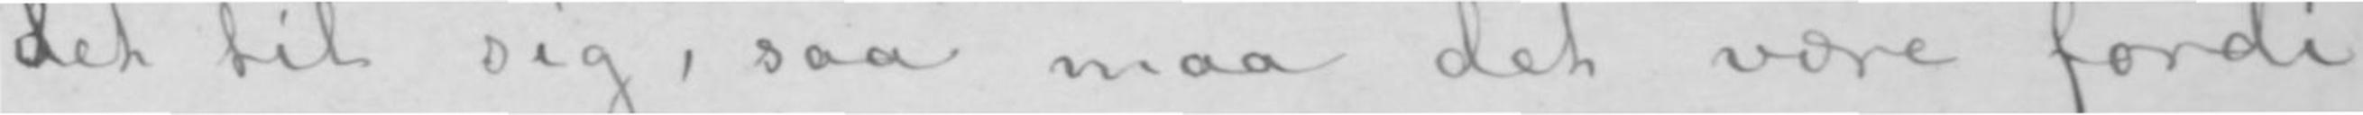det til sig, saa maa det være fordi
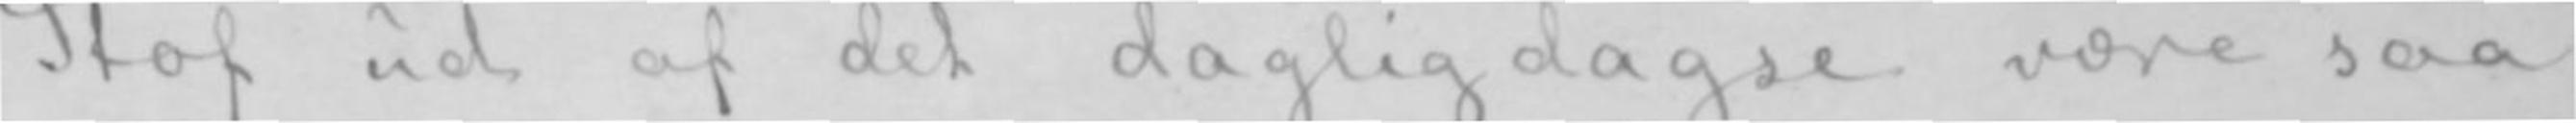Stof ud af det dagligdagse være saa
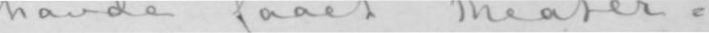havde faaet Theater-
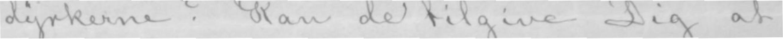dyrkerne? Kan de tilgive Dig at
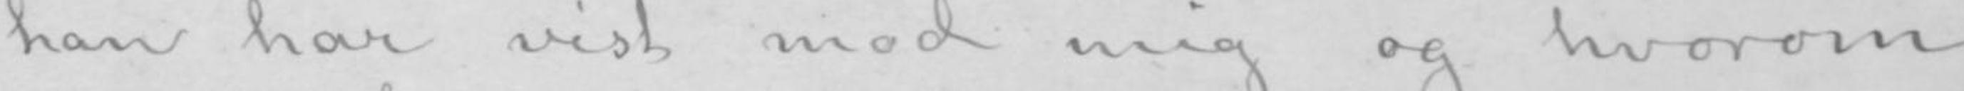han har vist mod mig og hvorom
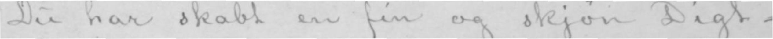Du har skabt en fin og skjøn Digt-
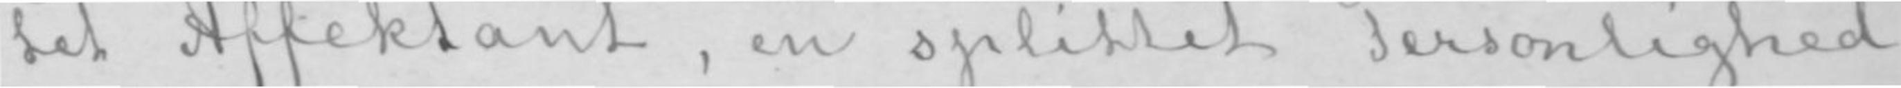tet Affektant, en splittet Personlighed,
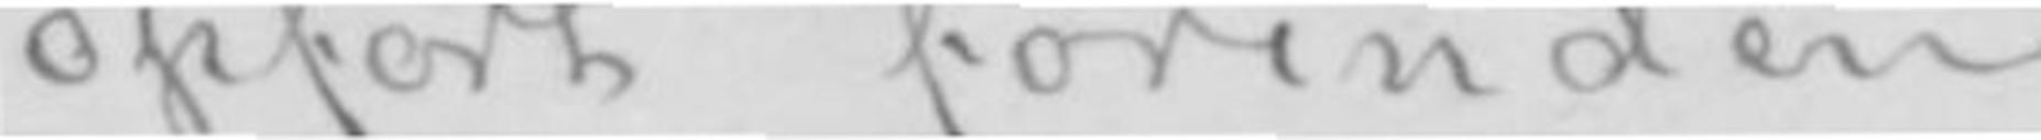opført forinden
